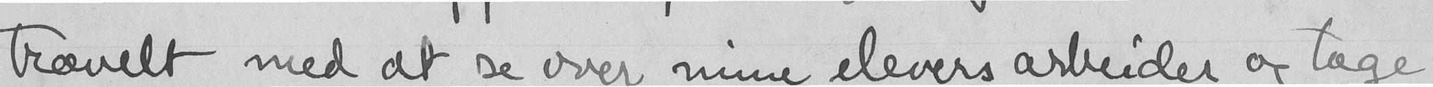travelt med at se over mine elevers arbeider og tage
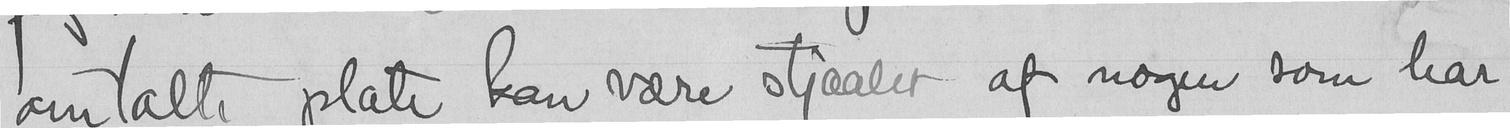omtalte plate kan være stjaalet af nogen som har
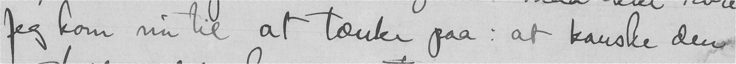Jeg kom nu til at tænke paa : at kanske den
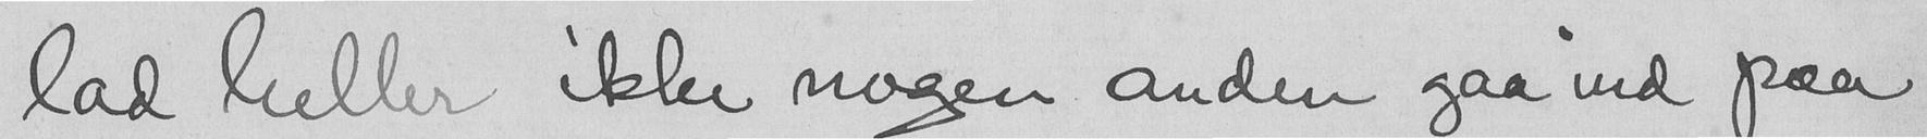lad heller ikke nogen anden gaa ind paa
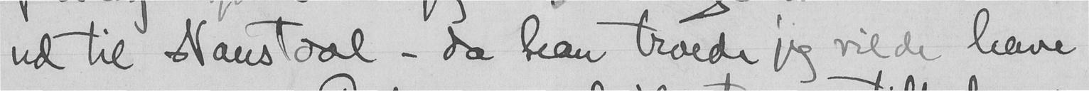ud til Naustdal - da han troede jeg vilde have
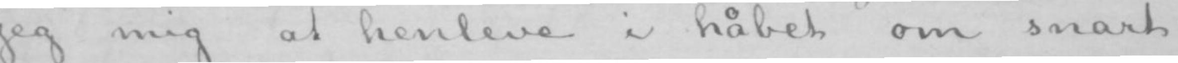jeg mig at henleve i håbet om snart
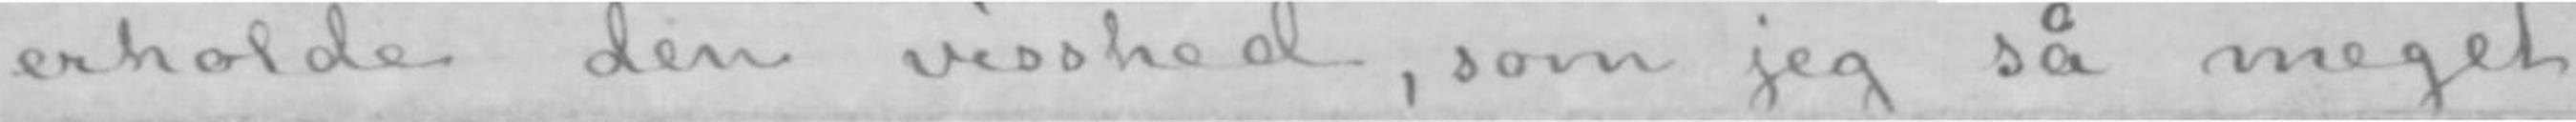erholde den visshed, som jeg så meget
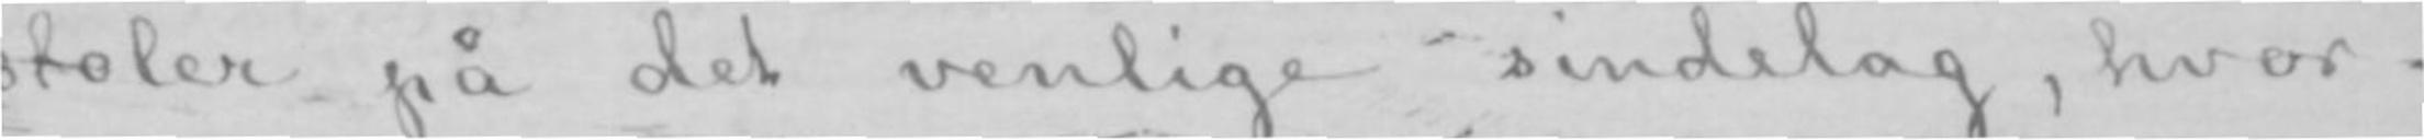stoler på det venlige sindelag, hvor-
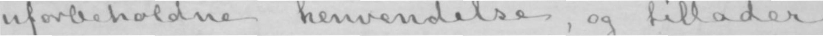uforbeholdne henvendelse, og tillader
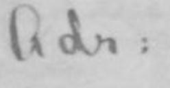Adr:
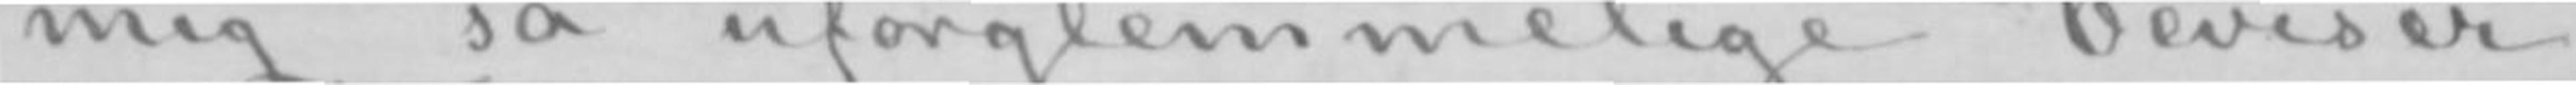mig så uforglemmelige beviser.
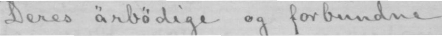Deres ärbödige og forbundne
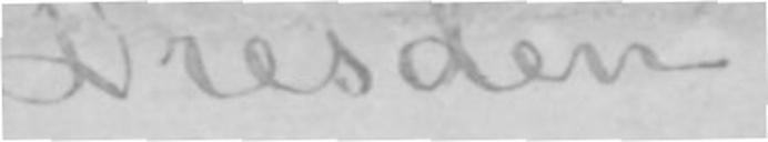Dresden.
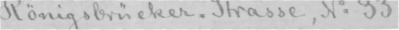Königsbrücker-Strasse, No 33.
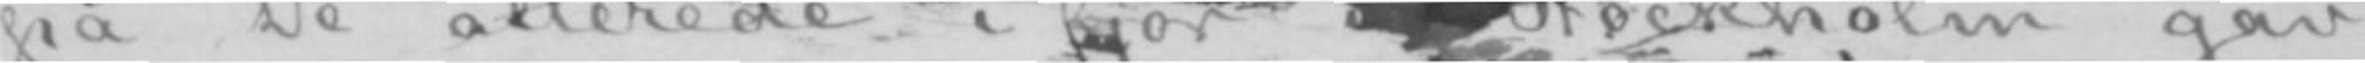på De allerede i fjor i Stockholm gav
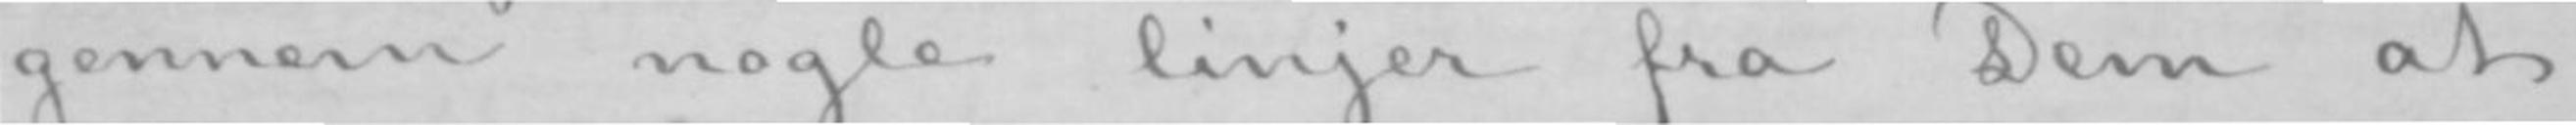gennem nogle linjer fra Dem at
